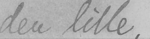den lille,
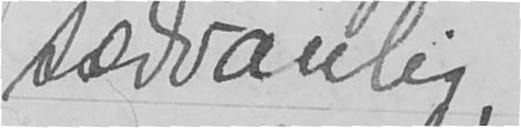sædvanlig,
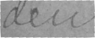den
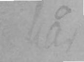hår-
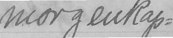morgenkap-
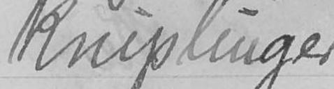kniplinger
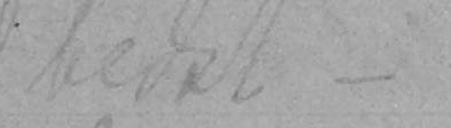bedst -
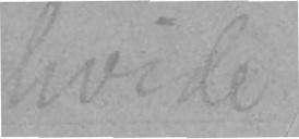hvide
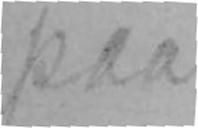paa
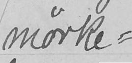mørke-
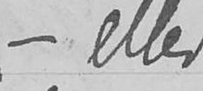- eller
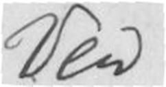Ven
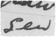sen
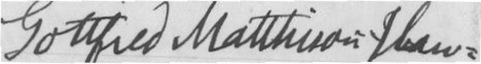Gottfred Matthison-Han-
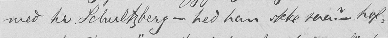med hr. Schultzberg – hed han ikke saa? – hof-
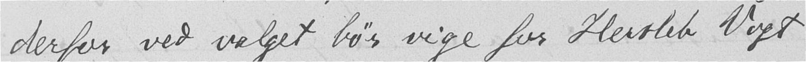derfor ved valget bør vige for Hersleb Vogt
